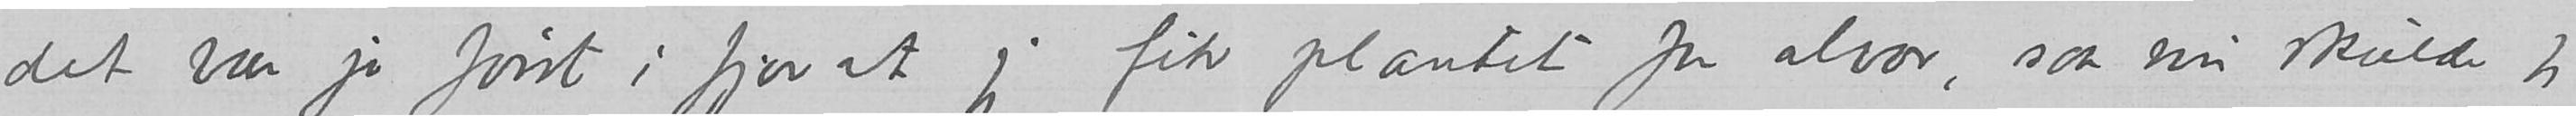det var jo først i fjor at jeg fik plantet for alvor, saa nu skulde jeg
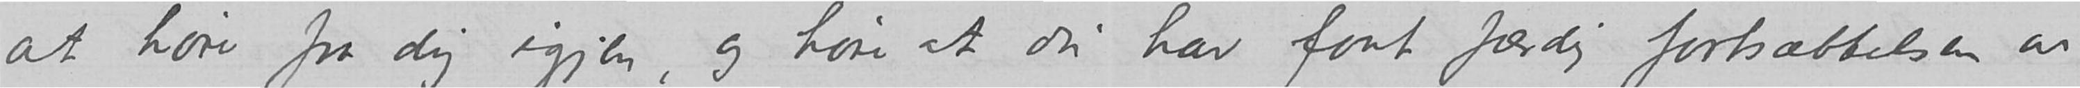at høre fra dig igjen, og høre at du har faat færdig fortsættelsen av
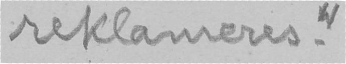reklameres."
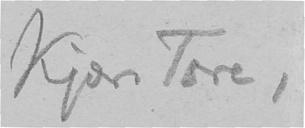Kjære Tore,
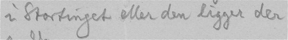i Stortinget eller den ligger der
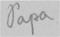Papa
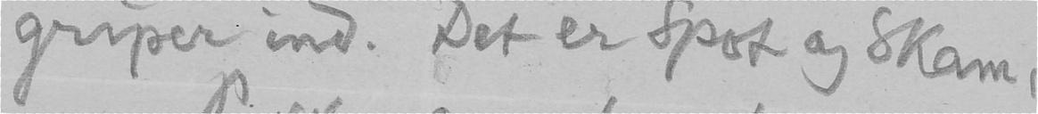griper ind. Det er Spot og Skam,
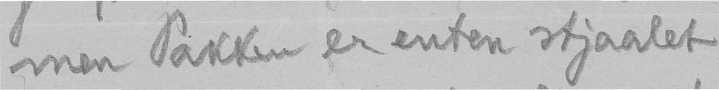men Pakken er enten stjaalet
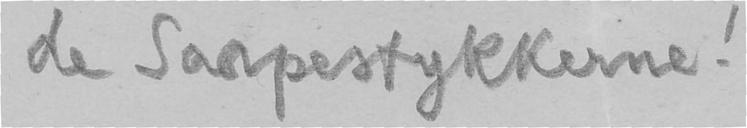de Saapestykkerne!
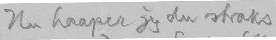Nu haaper jeg du straks
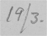19/3.
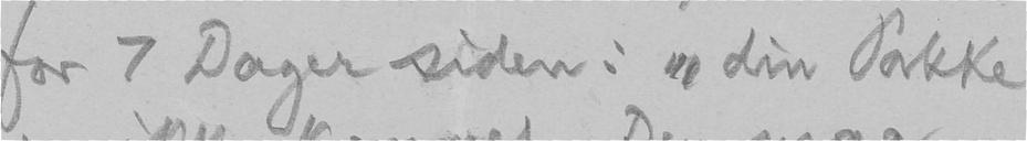for 7 Dager siden: "din Pakke
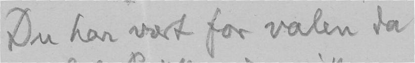Du har vært for valen da
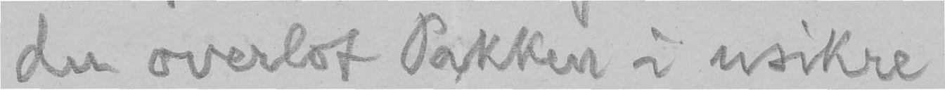du overlot Pakken i usikre
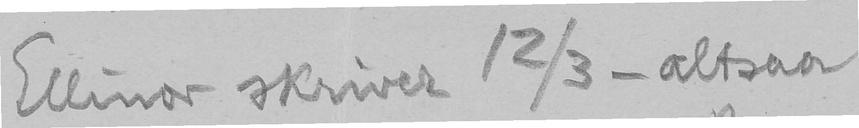Ellinor skriver 12/3 - altsaa
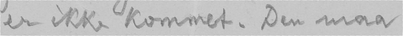er ikke kommet. Den maa
